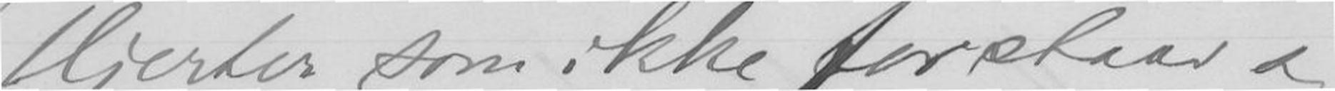Hjerter som ikke forstaar sig
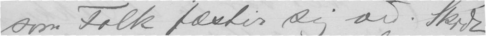som Folk fæster sig ved. Skider
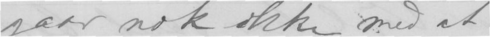gaar nok ikke med at
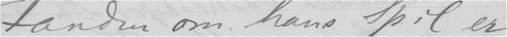Fanden om hans Spil er
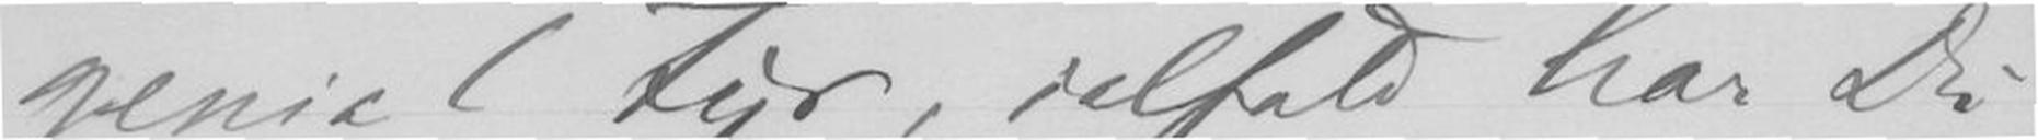genial Fyr, ialtfald har Du
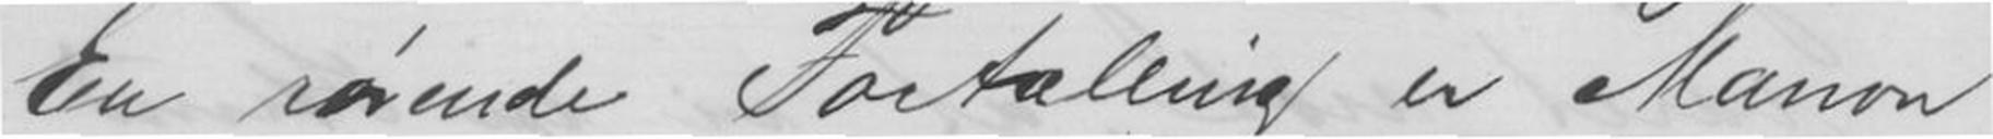En rørende Fortælling er Manon
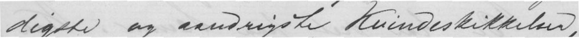digste og aandrigste Kvindeskikkelser,
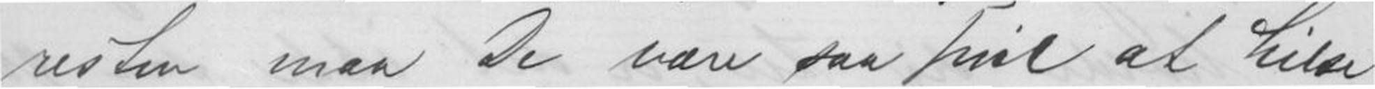resten maa De være saa snil at hilse
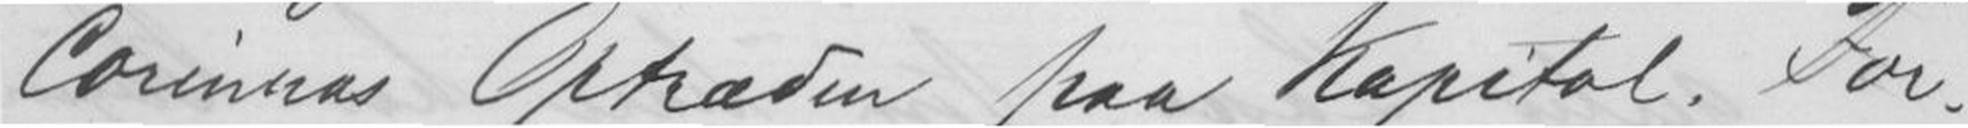Corinnas Optræden paa Kapitol. For-
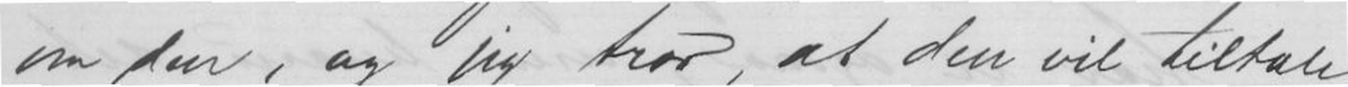om dur, og jeg tror, at den vil tiltale
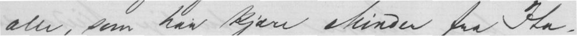alle, som har kjære Minder fra Ita-
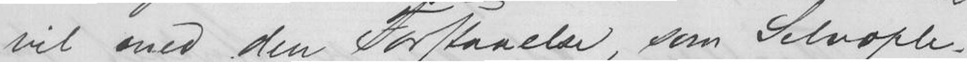vil med den Forstaaelse, som Selvople-
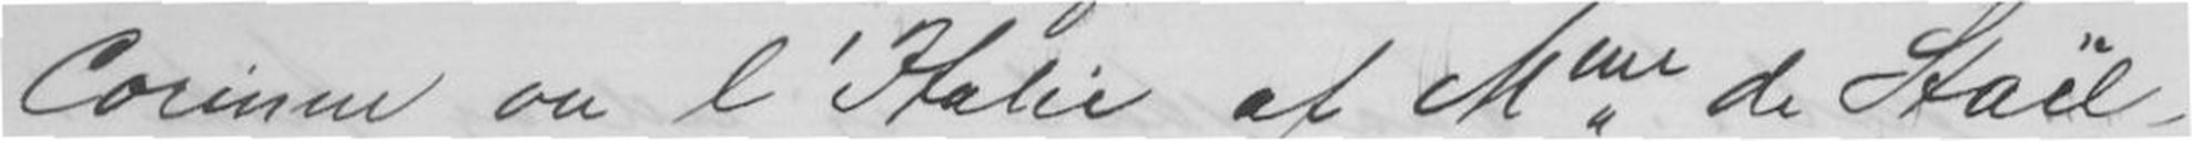Corinne on l'Italie af Mme de Stäel-
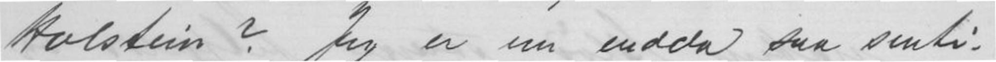Holstein? Jeg er nu endda saa senti-
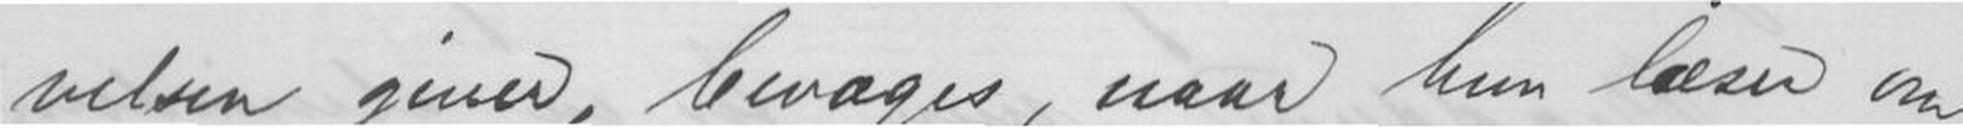velsen giver, bevæges, naar hun læser om
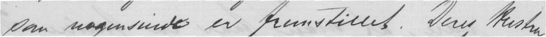som nogensinde er fremstillet. Deres Hustru
